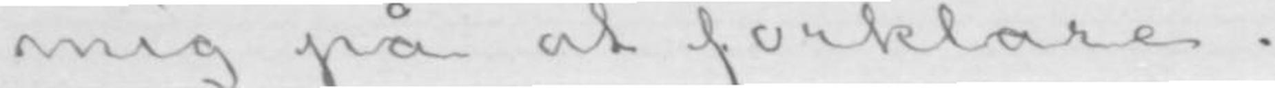mig på at forklare.
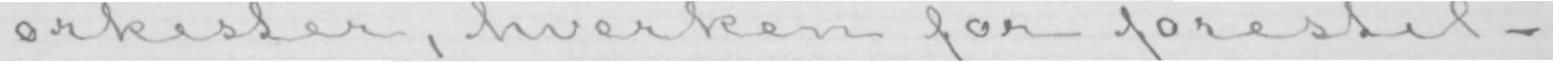orkester, hverken før forestil-
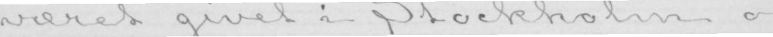været givet i Stockholm og
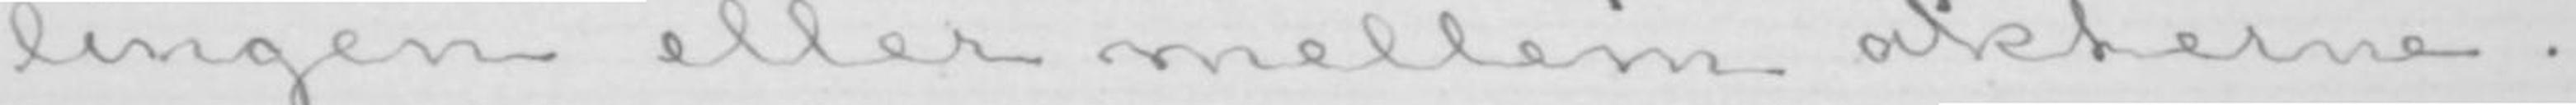lingen eller mellem akterne.
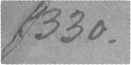330.
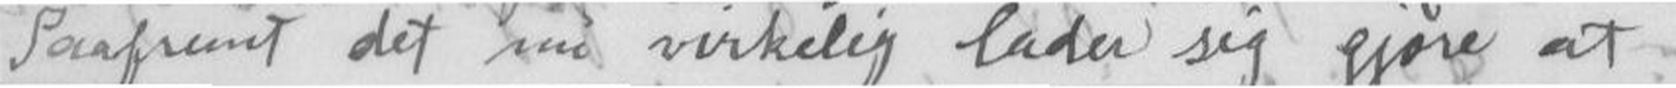Saafremt det nu virkelig lader sig gjøre at
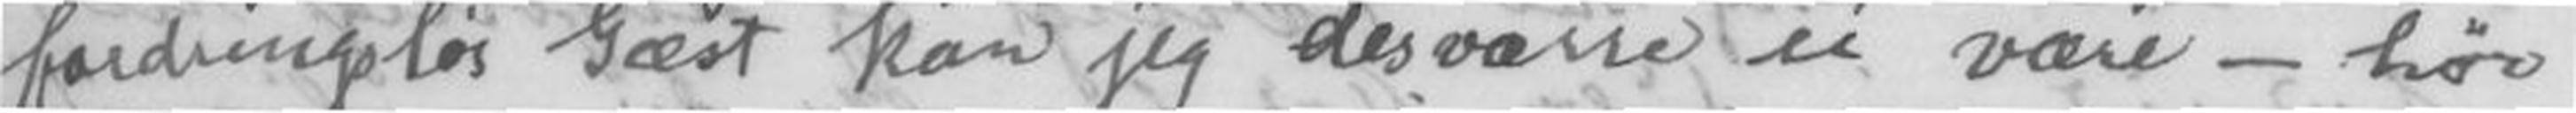fordringsløs Gæst kan jeg desværre ei være - hør
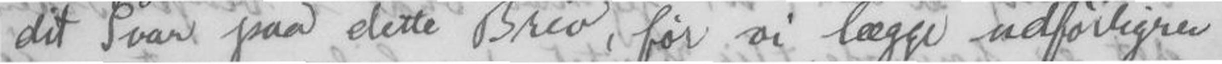dit Svar paa dette Brev, før vi lægge udførligere
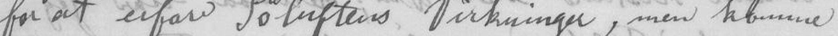for at erfare Søluftens Virkninger, men komme
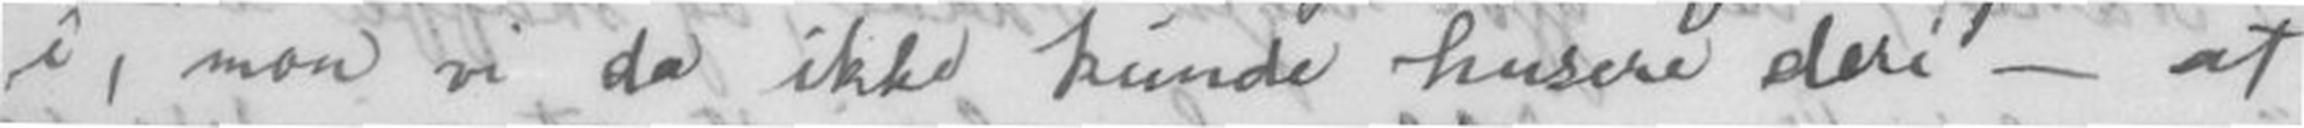i, mon vi da ikke kunde husere deri - at
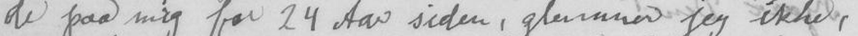de paa mig for 24 Aar siden, glemmer jeg ikke,
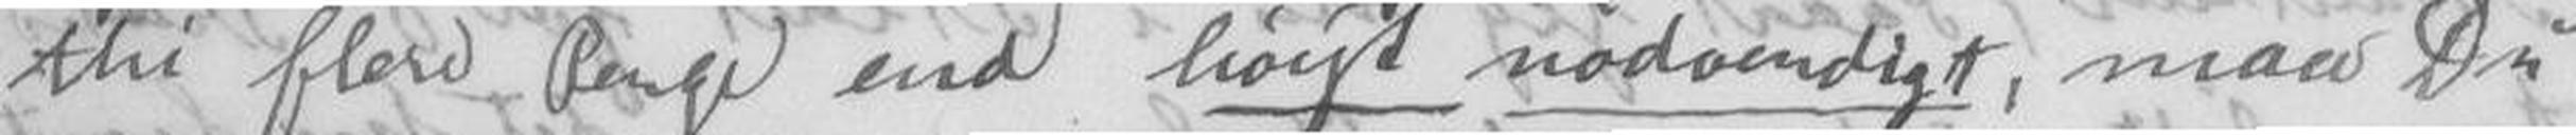thi flere Penge end høist nødvendigt, maa Du
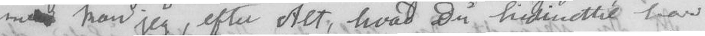nu kan jeg, efter Alt, hvad Du hidindtil hav
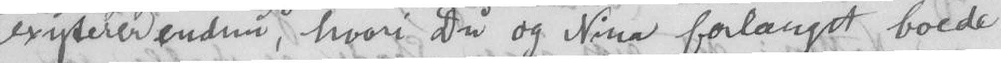existerer endnu, hvori Du og Nina forlanget boede
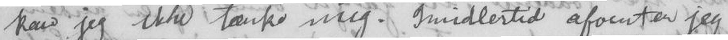kan jeg ikke tænke mig. Imidlertid afventer jeg
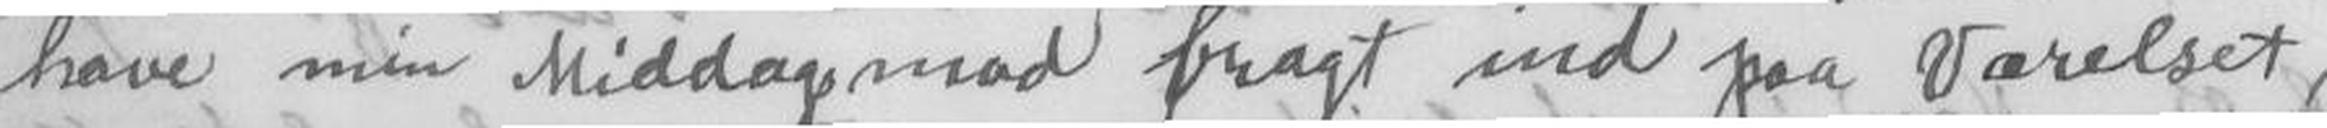have min Middagsmad bragt ind paa Værelset,
